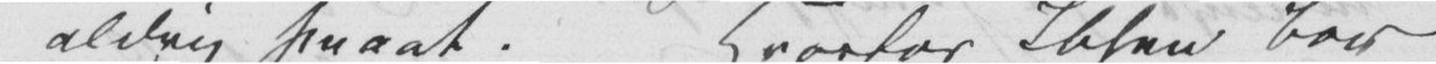aldrig smaat. Hvorfor Ibsen bor
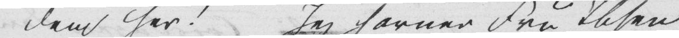Dem her! Jeg savner Fru Ibsen
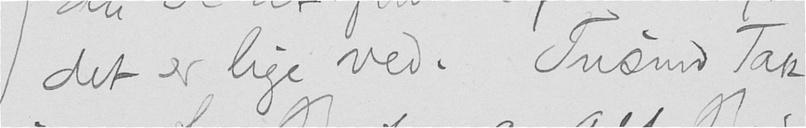det er lige ved. Tusind Tak
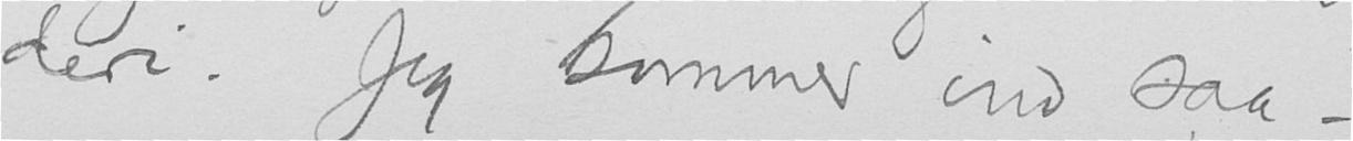deri. Jeg kommer ind saa-
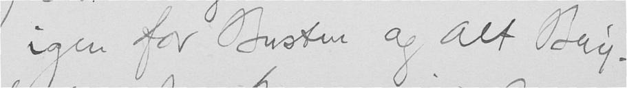igen for Busten og alt Bry-
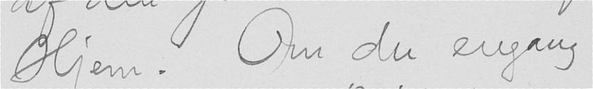Hjem. Om du engang
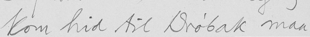kom hid til Drøbak maa
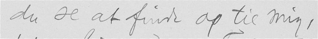du se at finde op til mig,
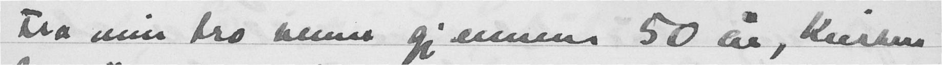Fra min tro venn gjennom 50 år, Kristen
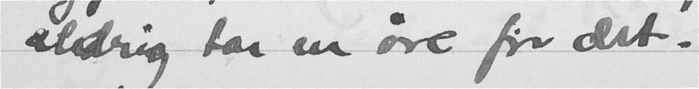aldrig tar en øre for det.
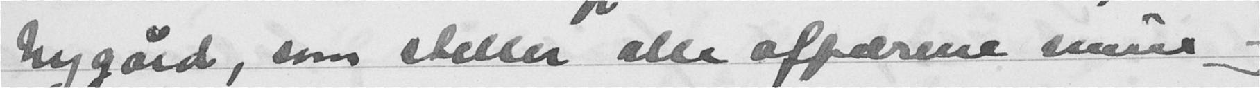Nygård, som stiller alle affærene mine og
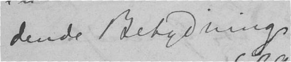dende Betydning.
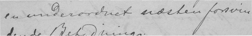en underordnet næsten forsvin-
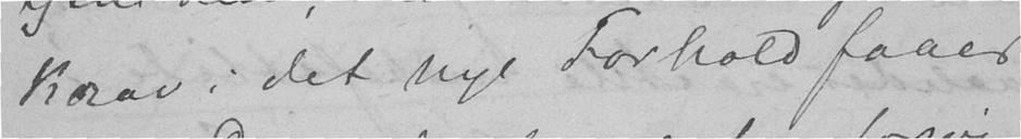Krav i det nye Forhold faaer
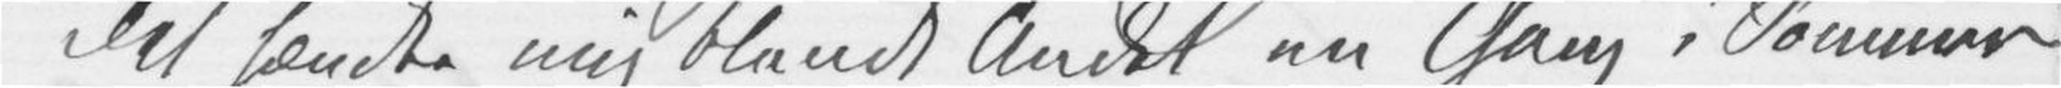Det hændte mig blandt Andet en Gang i Sommer
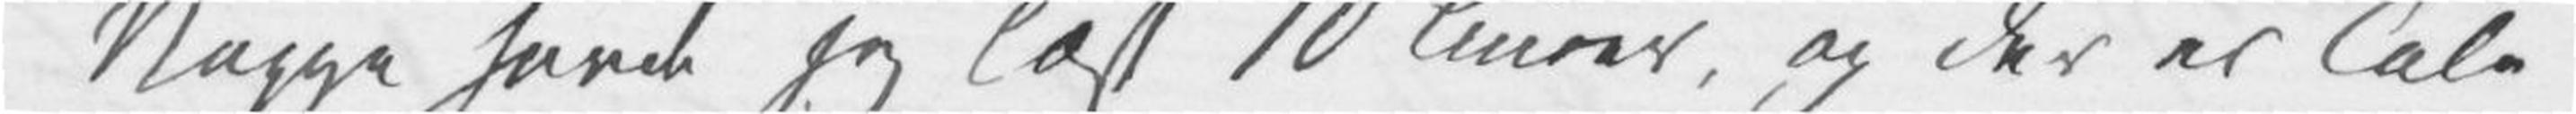Neppe havde jeg læst 10 Linier, og der er Tale
